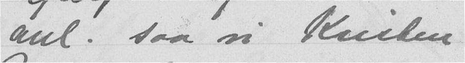anl. saa vi Kristen
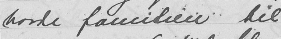havde familien til
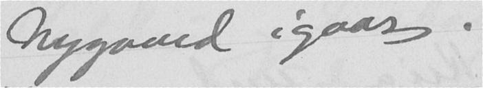Nygaard igaar.
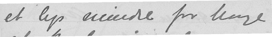et lys mindre for Norge
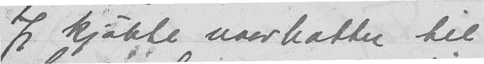Jeg kjøbte vaarhatter til
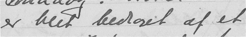er blit bedraget af et
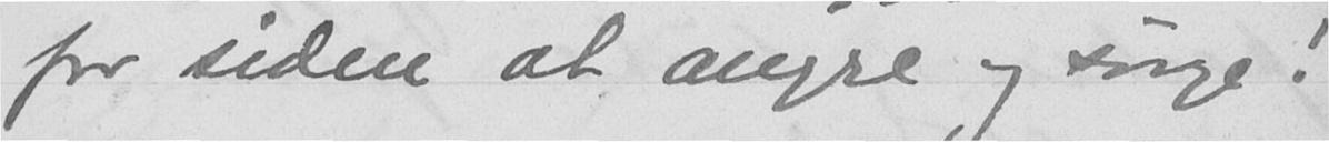for siden at angre og sørge!
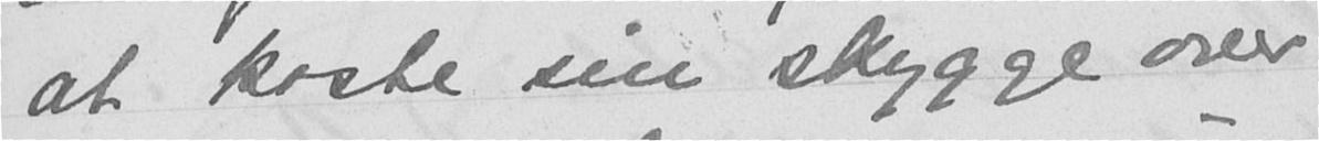at kaste sin skygge over
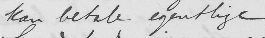kan betale egentlige
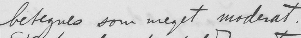betegnes som meget moderat.
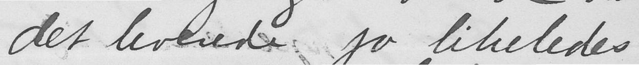det leverede jo likeledes
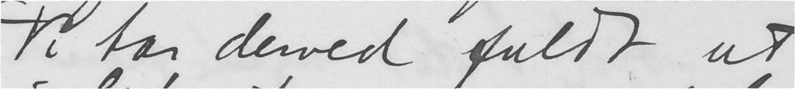Vi har derved fuldt ut
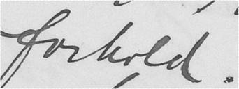forhold.
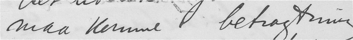maa komme i betragtning.
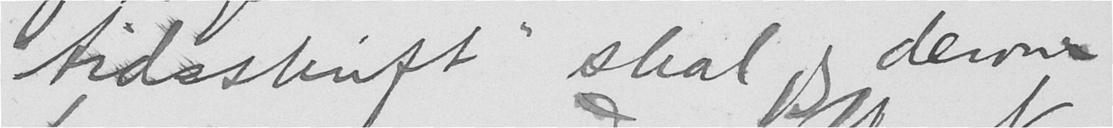tidsskrift" skal jeg derom
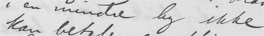i en mindre by ikke
